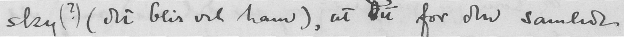sky(?) (det blir vel ham), at du for den samlede
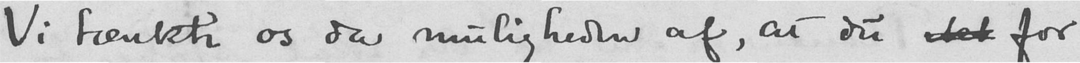Vi tænkte os da muligheden af, at du ikke for
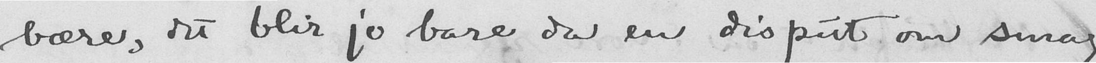bære, det blir jo bare da en disput om smag
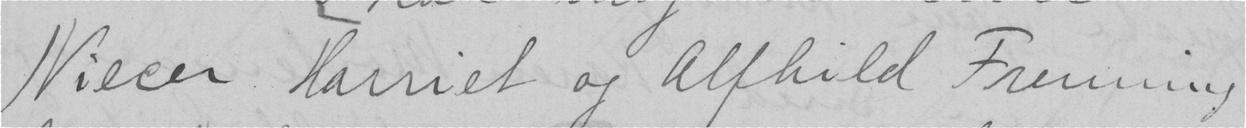Niecer Harriet og Alfhild Frenning
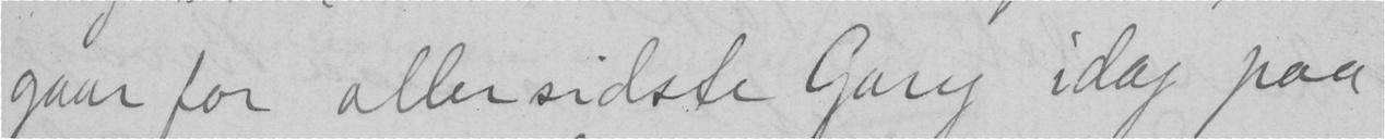gaar for aller sidste Gang idag paa
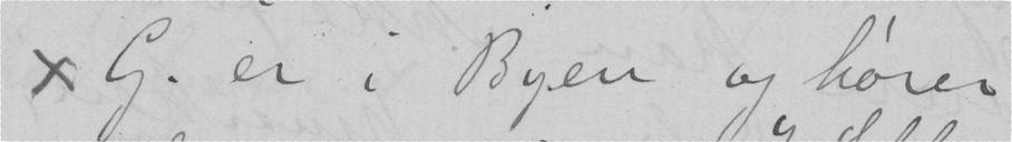x G. er i Byen og hører
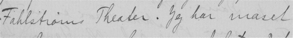Fahlstrøm Theater. Jeg har maset
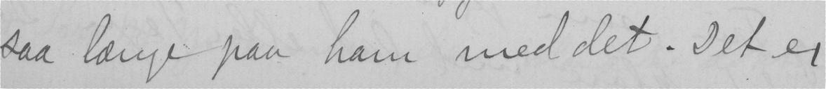saa længe paa ham med det. Det er
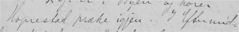Hognestad præke igjen. I eftermid-
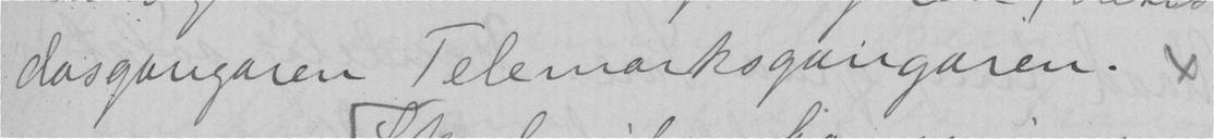dasgangaren Telemarksgangaren. x
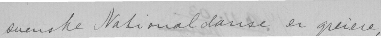svenske Nationaldanse er greiere,
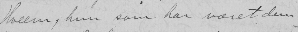Hveem, hun som har været dem
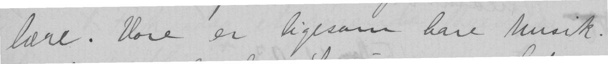lære. Vore er ligesom bare musik.
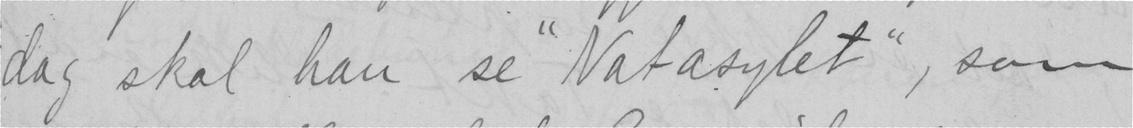dag skal han se "Natasylet", som
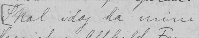Skal idag ha mine
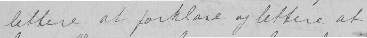lettere at forklare og lettere at
